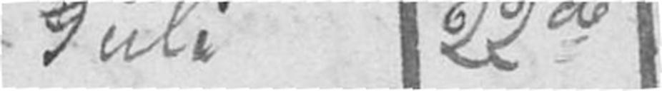Juli 22de
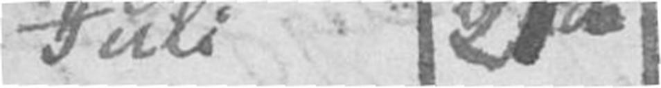Juli 21de
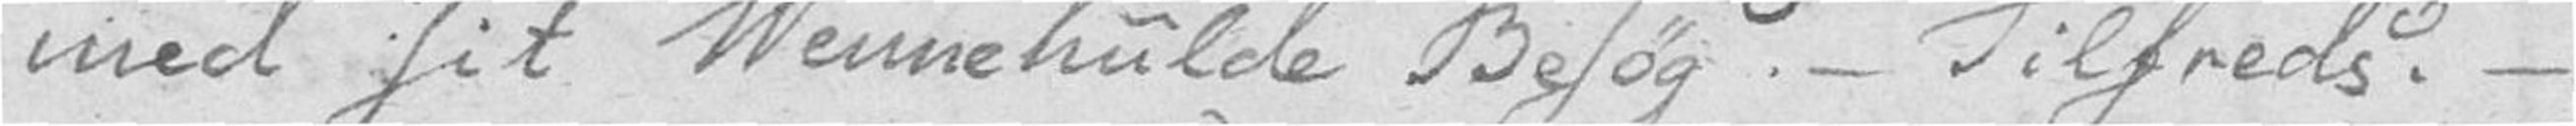med sit Vennehulde Besøg. – Tilfreds. –
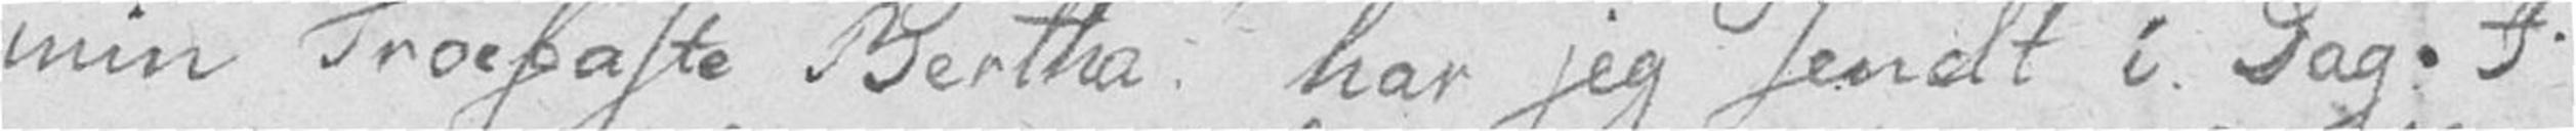min troefaste Bertha! har jeg sendt i Dag. I
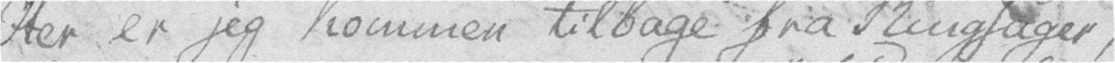Her er jeg kommen tilbage fra Ringsager,
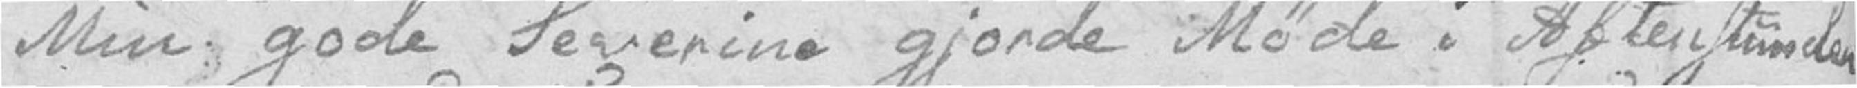Min gode Severine gjorde Møde i Aftenstunden
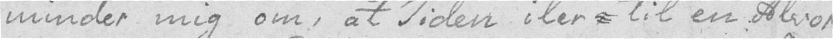minder mig om, at Tiden iler til en Alvor
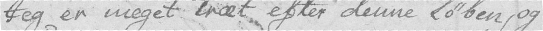Jeg er meget træt efter denne Løben, og
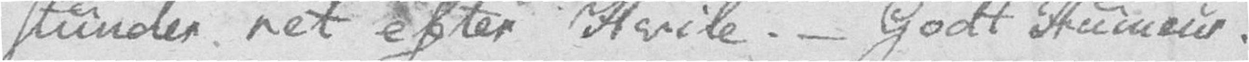stunder ret efter Hvile. – Godt Humeur.
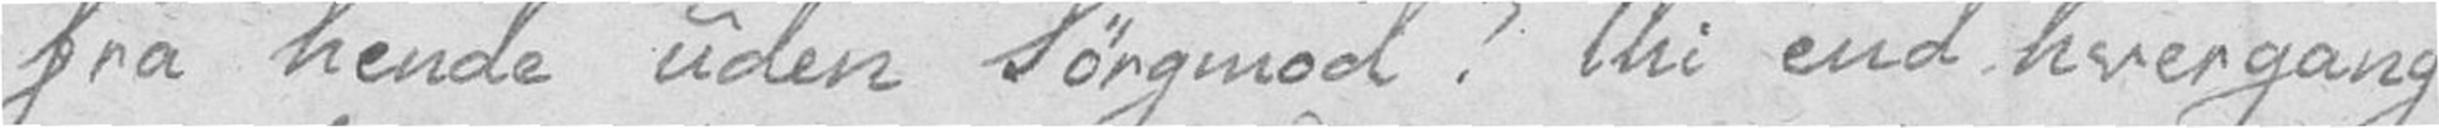fra hende uden Sørgmod? thi end hvergang
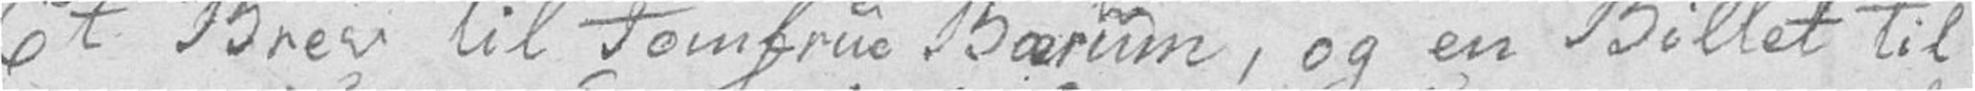Et Brev til Jomfrue Bærum, og en Billet til
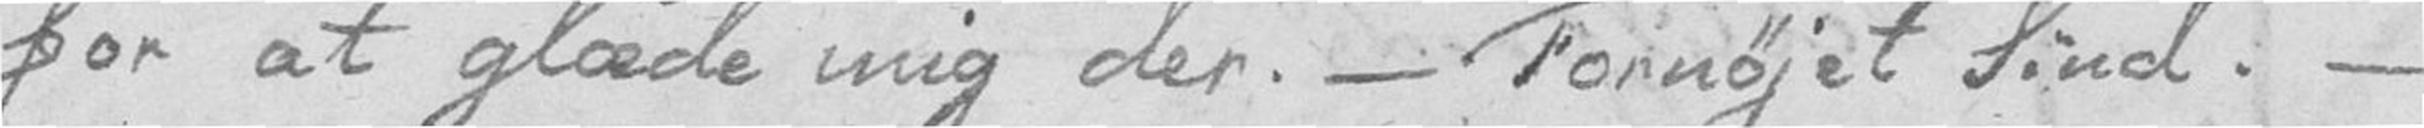for at glæde mig der. – Fornøjet Sind. –
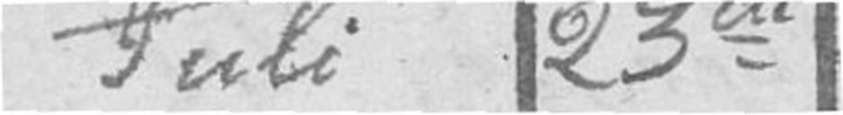Juli 23de
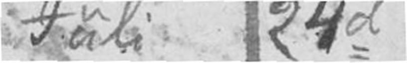Juli 24d
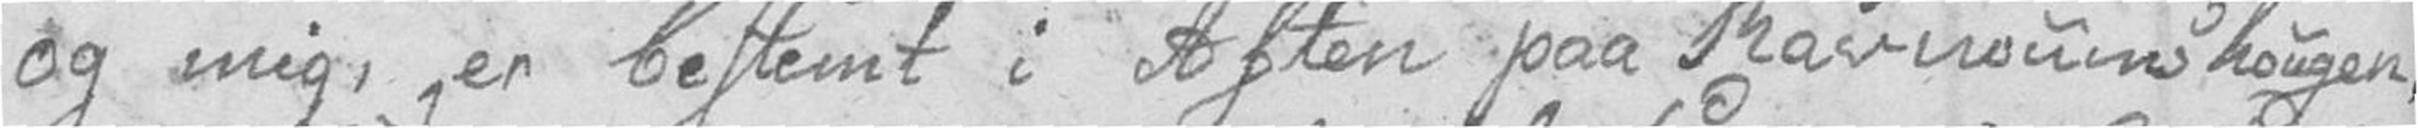og mig, er bestemt i Aften paa Ravnoumshougen.
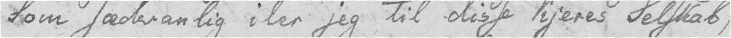Som sædvanlig iler jeg til disse kjeres Selskab,
